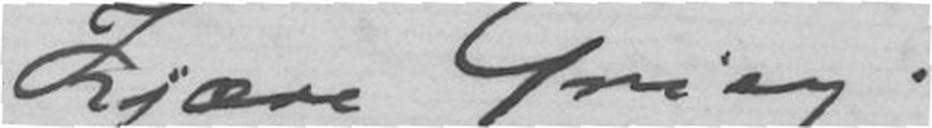Kjære Grieg!
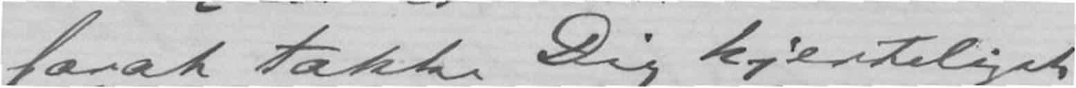forat takka Dig hjerteligst
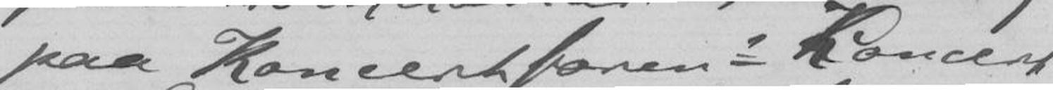paa Koncertforens Concert
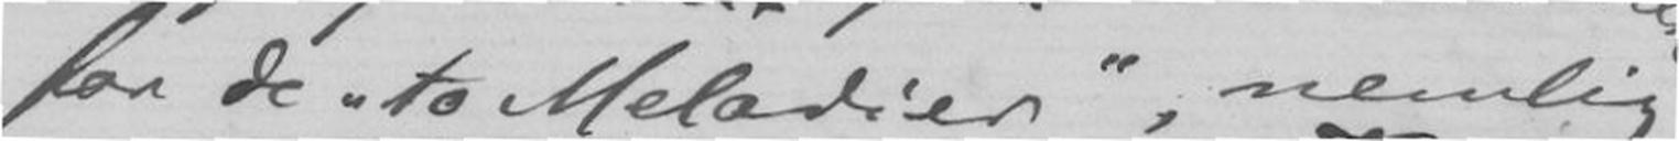for de to Melodier, nemlig
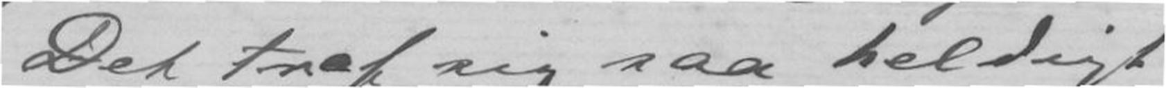Det traf sig saa heldigt
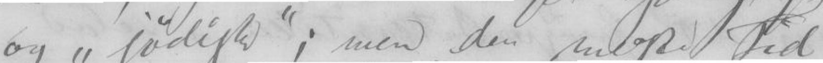og jødisk; men den meste Tid
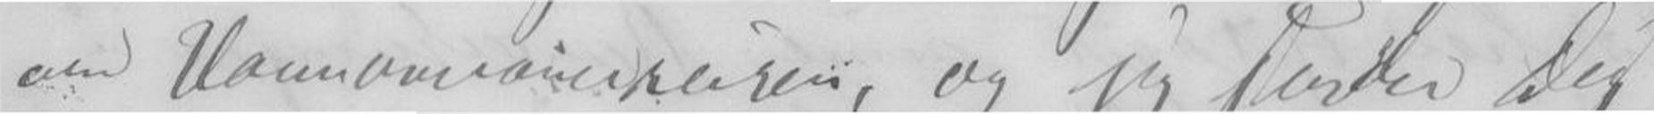om Hannoveranerreisen, og jeg sender Dig
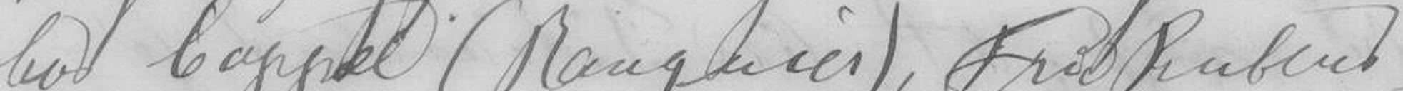hos Coppel (Banquier), Fru Rubens
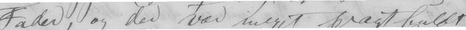Fader, og den var meget pragtfuldt
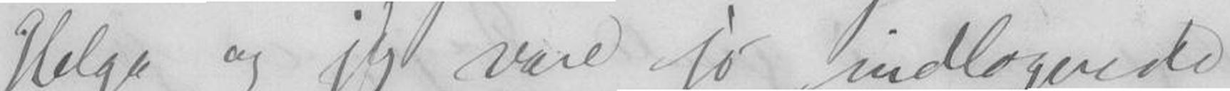Helga og jeg var jo indlogerede
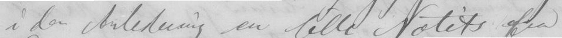i den Anledning en lille Notits fra
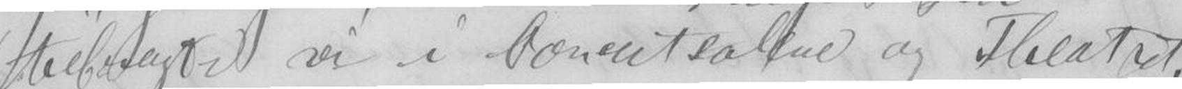tilbragte vi i Concertsalene og Theatret.
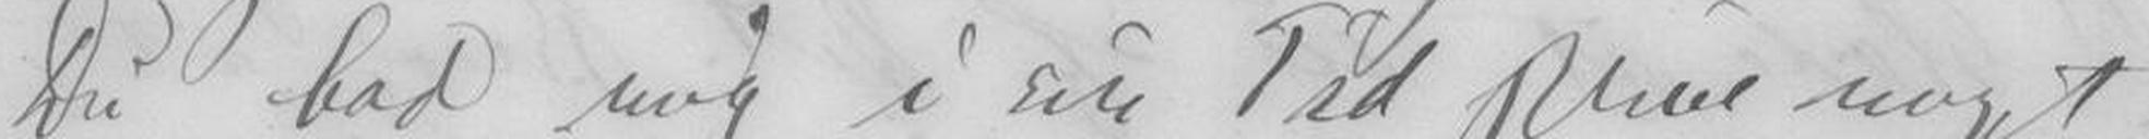Du bad mig i sin Tid skrive noget
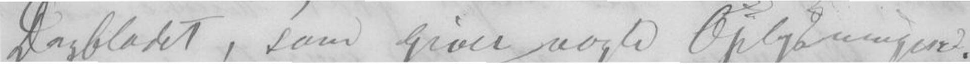Dagbladet, som giver nogle Oplysninger.
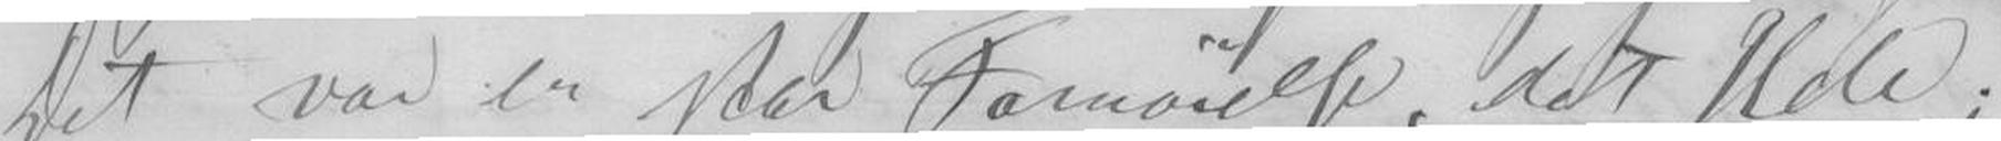Det var en stor Fornøielse, det Hele;
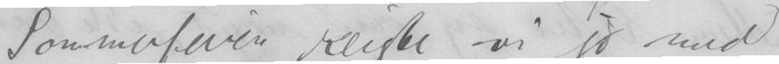Sommerferien reiste vi jo med
